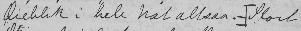Øieblik i hele Nat altsaa. Stort
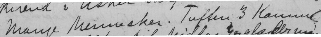mange mennesker. Tuften 3 Kamme-
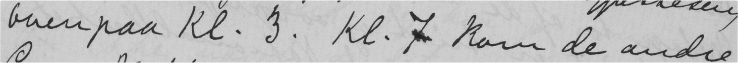ovenpaa Kl. 3. Kl. 7 kom de andre
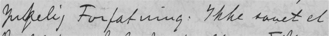Ynkelig Forfatning. Ikke sovet et
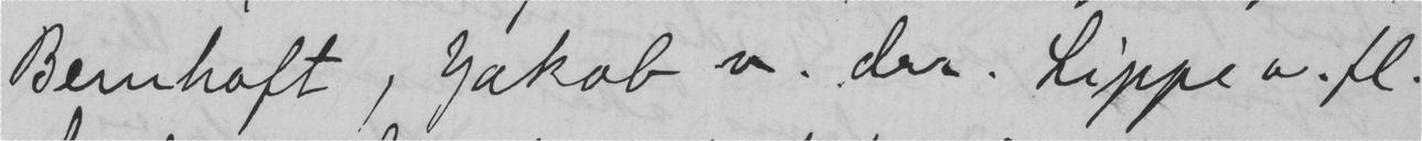Bernhoft, Jakob v. der. Lippe v. fl.
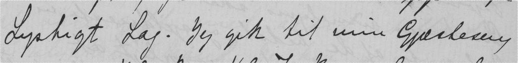Lystigt Lag. Jeg gik til min Gjæsteseng
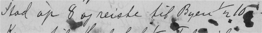Stod op 8 og reiste til Byen 1/2 10  .
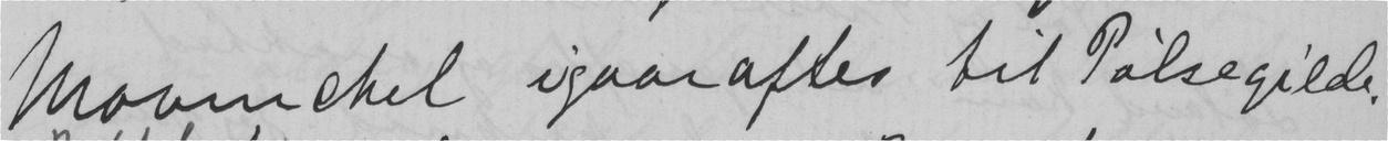Movinckel igaaraftes til Pølsegilde.
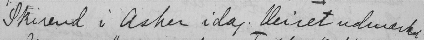Skirend i Asker idag. Veiret udmærket
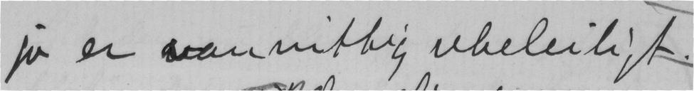jo er vanvittig ubeleiligt.
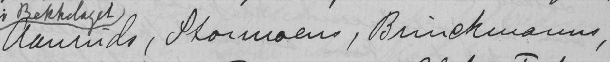Aansuds, Stormoens, Brinckmanns,
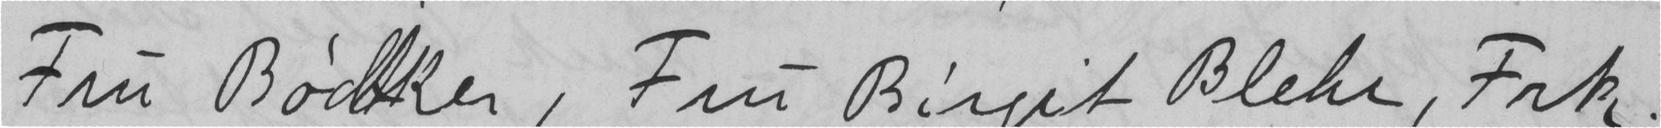Fru Bødker, Fru Birgit Blehr, Frk.
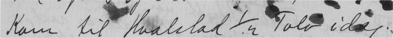Kom til Hvalstad 1/2 Tols idag.
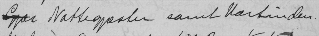Nattegjæster samt Værtinden.
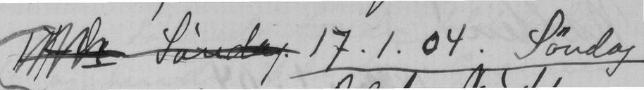17.1.04. Søndag
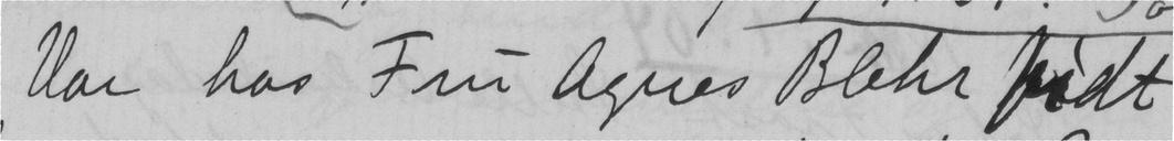Var hos Fru Agnes Blehr født
